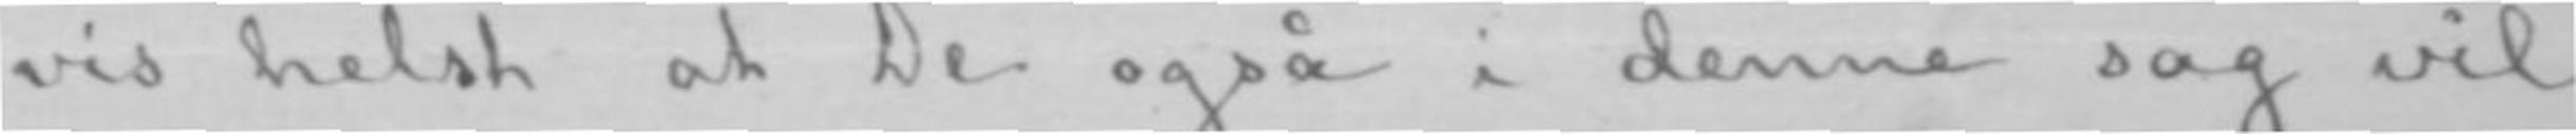vis helst at De også i denne sag vil
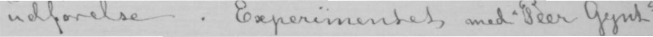udførelse. Experimentet med «Peer Gynt»
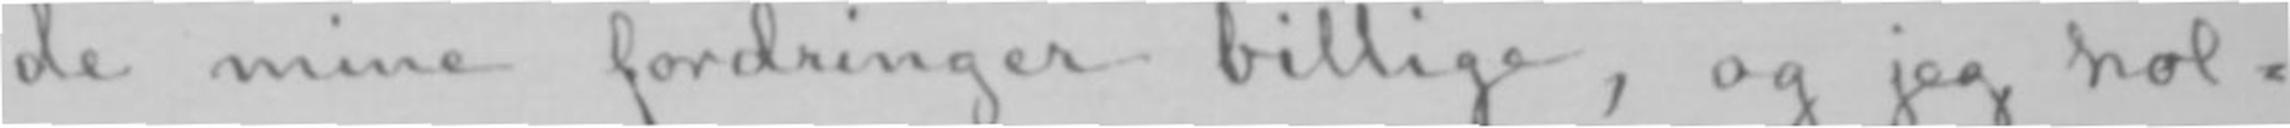de mine fordringer billige, og jeg hol-
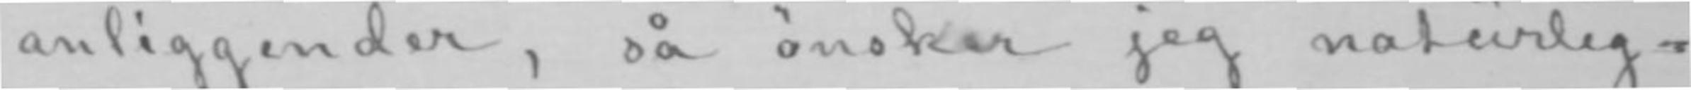anliggender, så ønsker jeg naturlig-
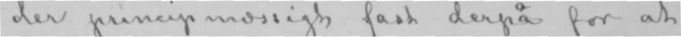der principmæssigt fast derpå for at
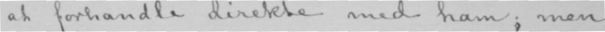at forhandle direkte med ham; men
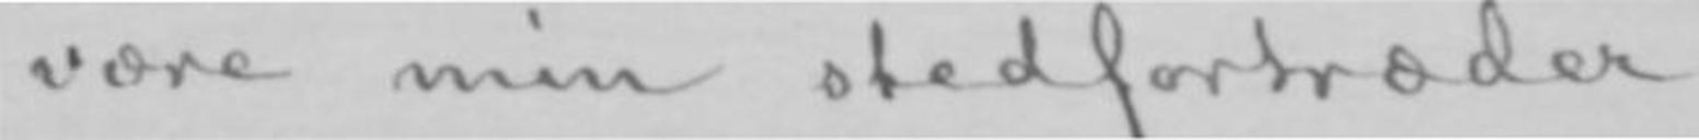være min stedfortræder.
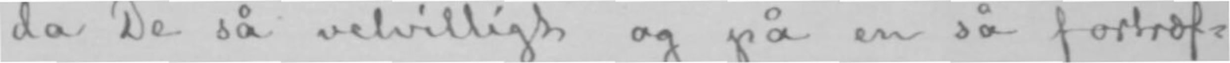da De så velvilligt og på en så fortræf-
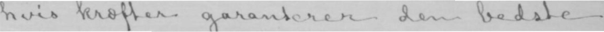hvis kræfter garanterer den bedste
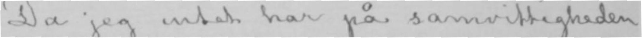Da jeg intet har på samvittigheden
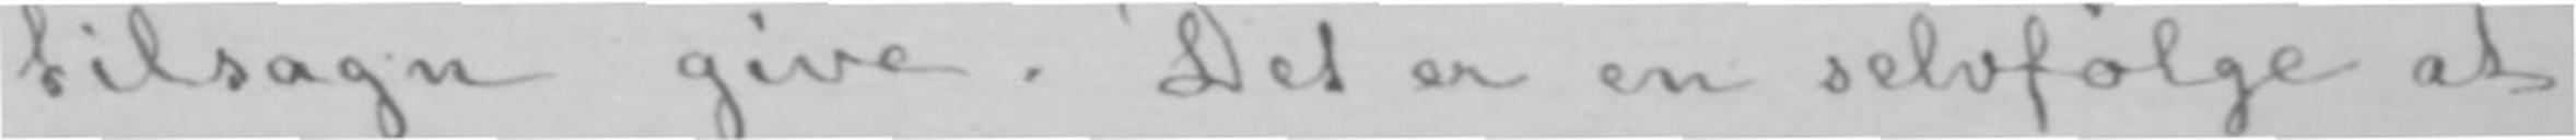tilsagn give. Det er en selvfølge at
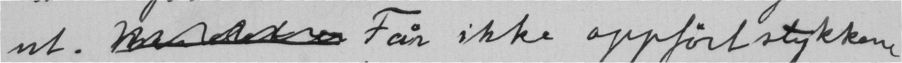ut.  Får ikke oppført stykkene
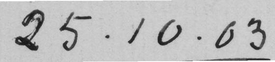25.10.03
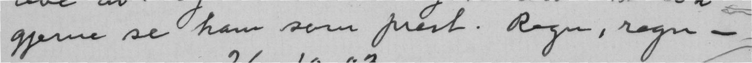gjerne se ham som prest. Regn, regn -
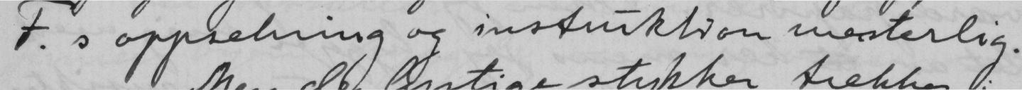F. s oppsetning og instruktion mesterlig.
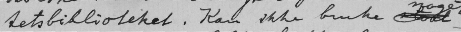tetsbiblioteket. Kan ikke bruke
